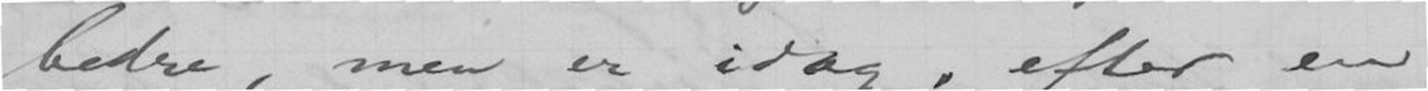bedre, men er idag, efter en
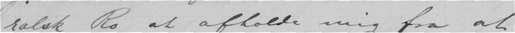ralsk Ro at afholde mig fra at
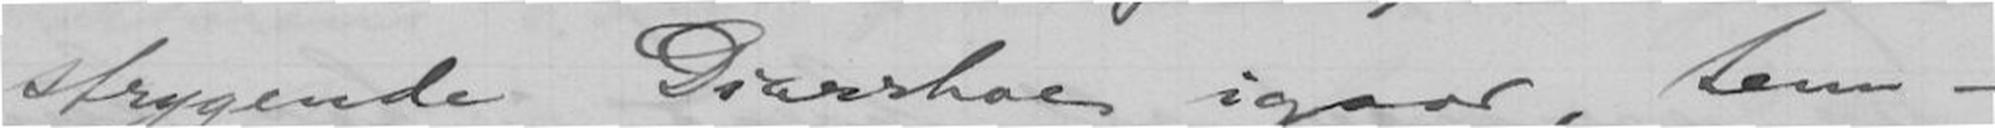strygende Diarrhoe igaar, tem-
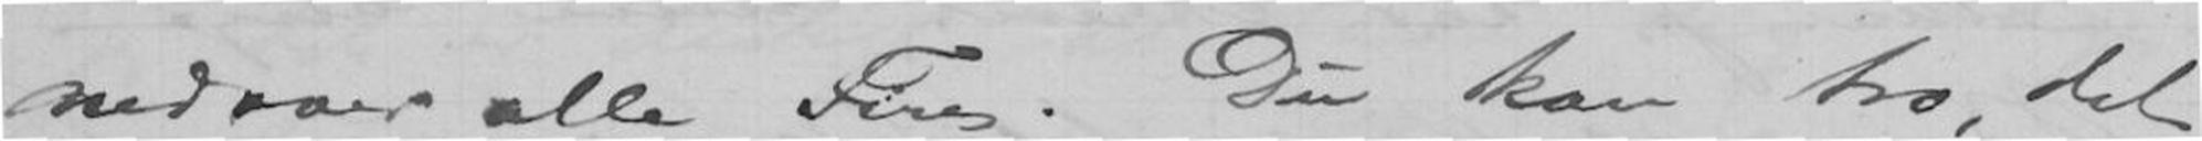nedover alle Fire. Du kan tro, det
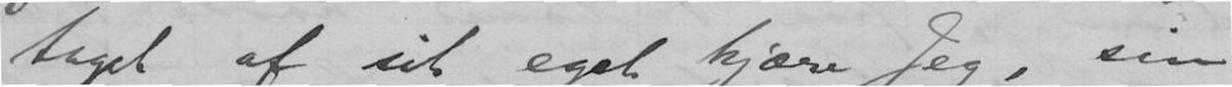taget af sit eget kjære Jeg, sin
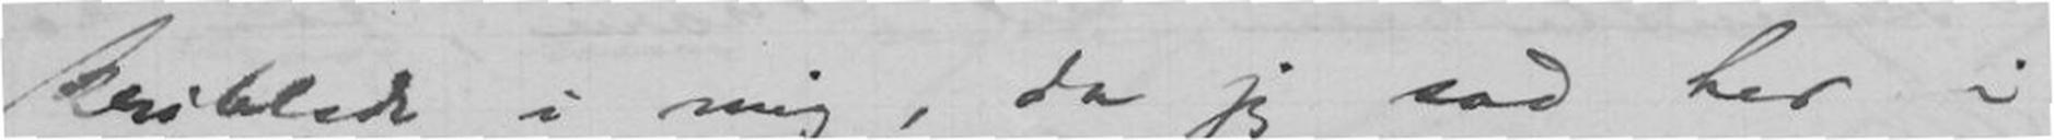kriblede i mig, da jeg sad her i
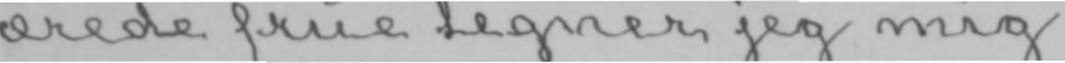ærede frue tegner jeg mig
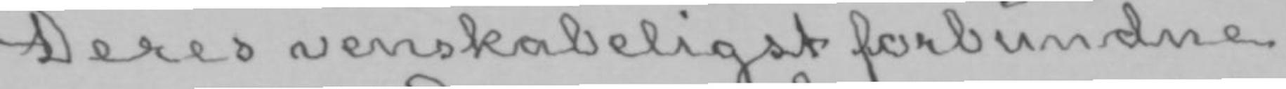Deres venskabeligst forbundne
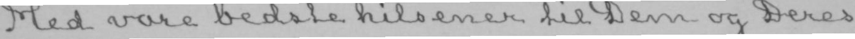Med vore bedste hilsener til Dem og Deres
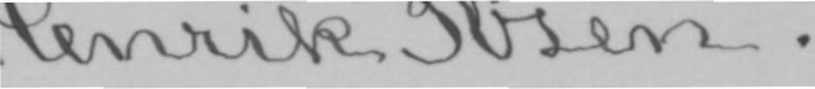Henrik Ibsen.
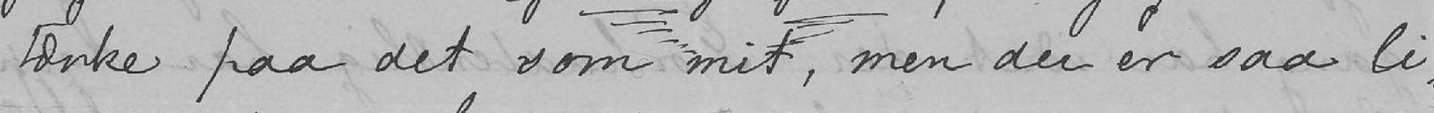tænke paa det som mit, men der er saa li-
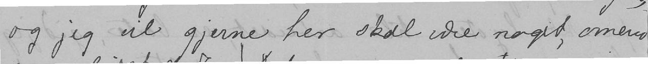og jeg vil gjerne her skal være noget, omend
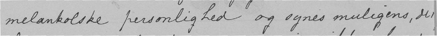melankolske personlighed og synes muligens, der
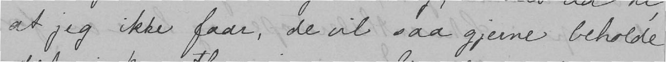at jeg ikke faar, de vil saa gjerne beholde
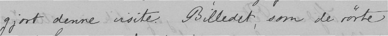gjort denne visite. Billedet, som de rørte
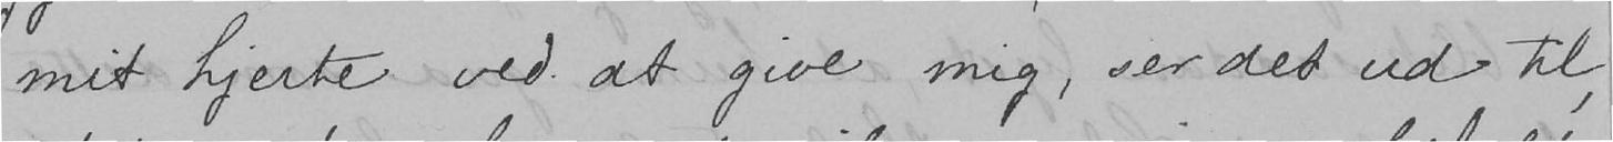mit hjerte ved at give mig, ser det ud til,
